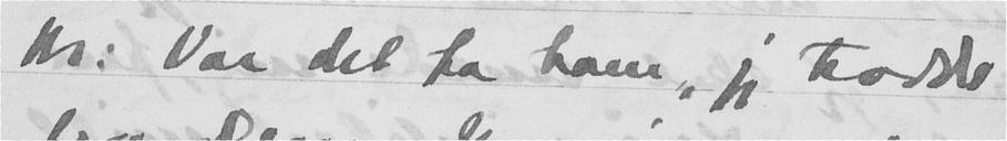M: Var det fra ham, jeg trodde
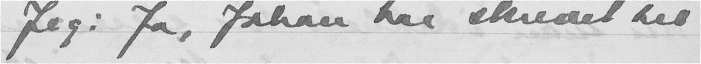Jeg: Ja, Johan har skrevet til
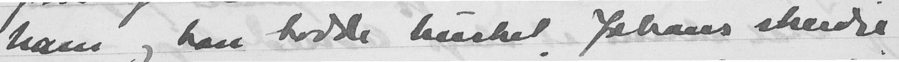ham og han hadde husket Johans iherdige
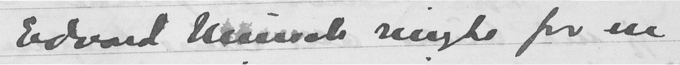Edvard Munch ringte for en
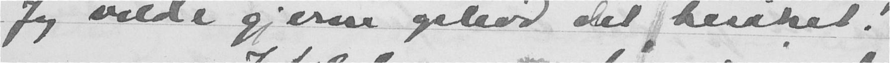Jeg vilde gjerne oplevd det besøket!
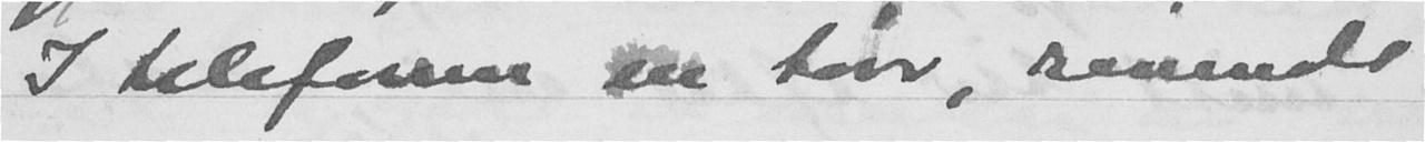I telefonen en tørr, svinnende
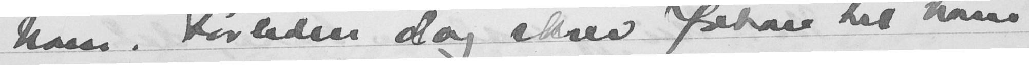ham. Forleden dag skrev Johan til ham
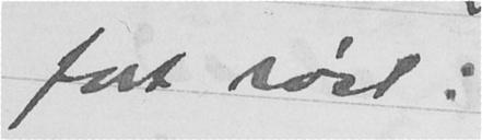fast røst:
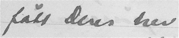fått Deres brev
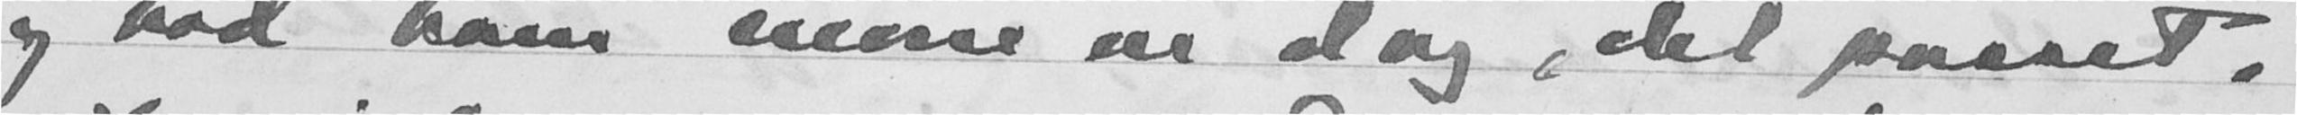og bad ham nevne en dag, det passet.
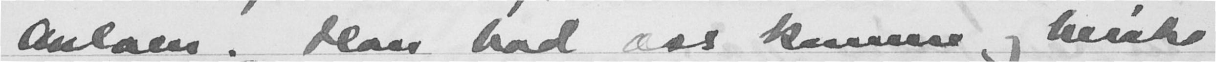Aulaen. Han bad oss komme og besøke
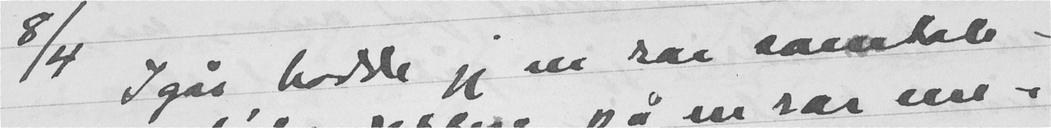8/4 Igår hadde jeg en rar samtale -
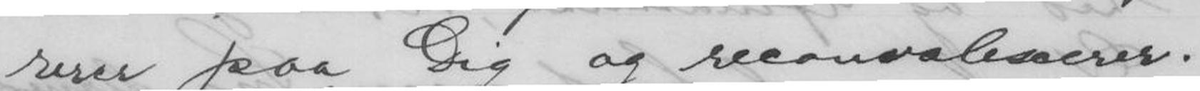rerer paa Dig og reconvalescerer.
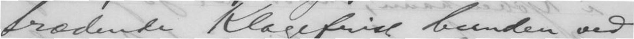trædende Klagefrist bunden ved
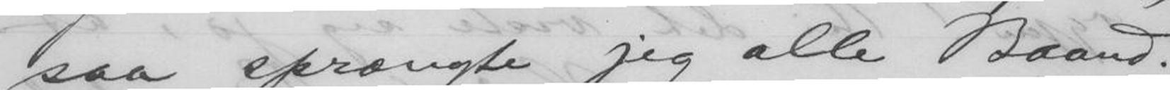saa sprængte jeg alle Baand.
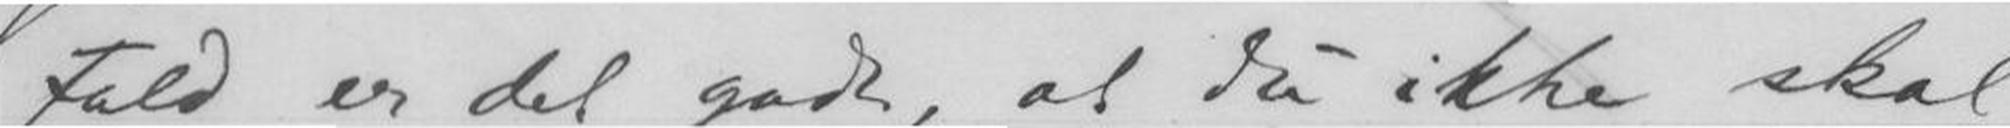Fald er det godt, at du ikke skal
